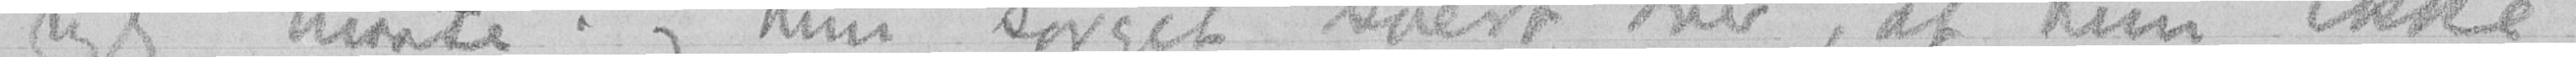rigtig maate; og hun sørget svært over, at hun ikke
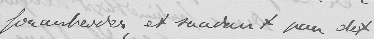forarbeider, et saadant paa det
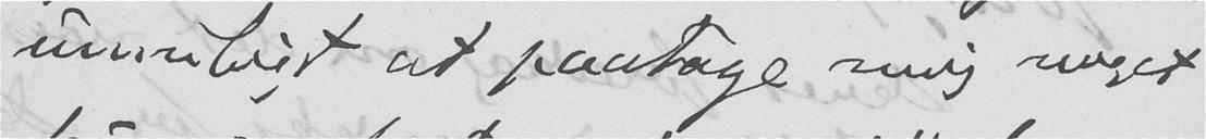umuligt at paatage mig noget
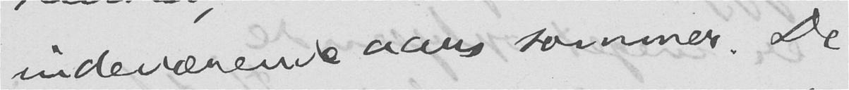indeværende aars sommer. De
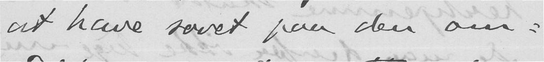at have sovet paa den om-
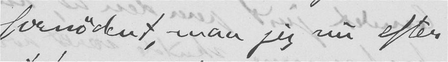fornødent, maa jeg nu efter
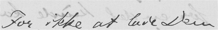For ikke at lade Dem
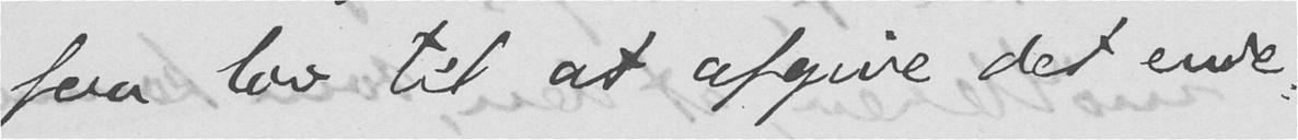faa lov til at afgive det ende-
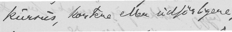kursus, kortere eller udførligere,
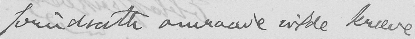forudsatte omraade vilde kræve,
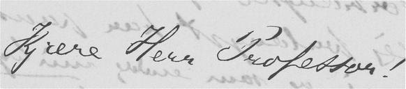Kjære Herr Professor!
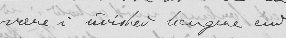være i uvished længere end
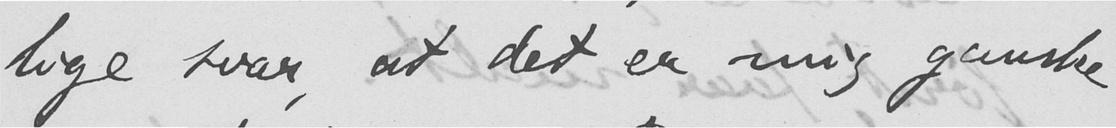lige svar, at det er mig ganske
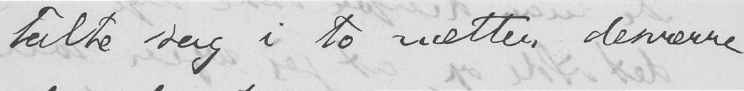talte sag i to nætter desværre
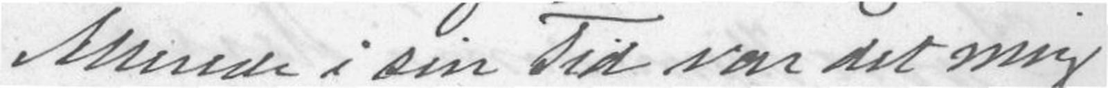Allerede i sin Tid var det mig
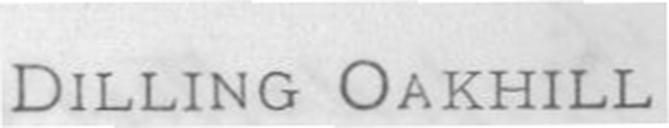DILLING OAKHILL
